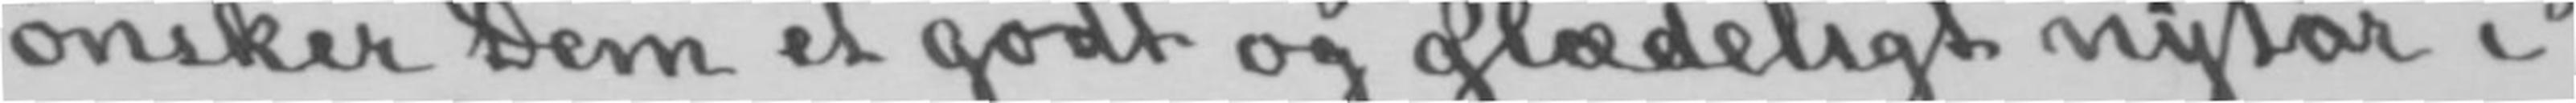ønsker Dem et godt og glædeligt nytår i
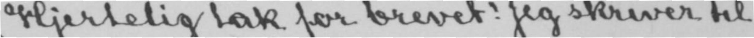Hjertelig tak for brevet! Jeg skriver til
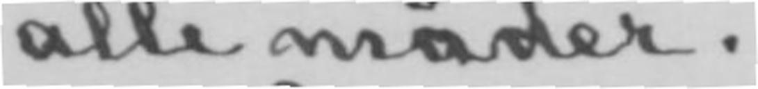alle måder.
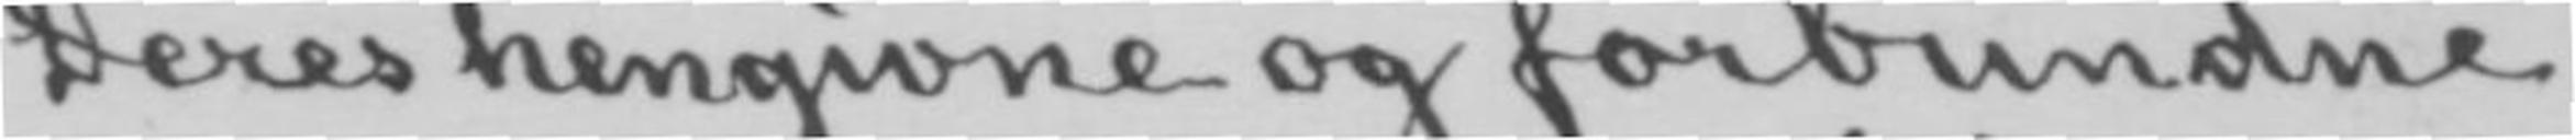Deres hengivne og forbundne
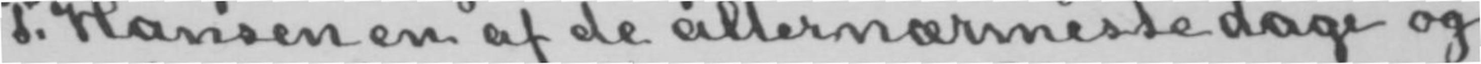P. Hansen en af de allernærmeste dage og
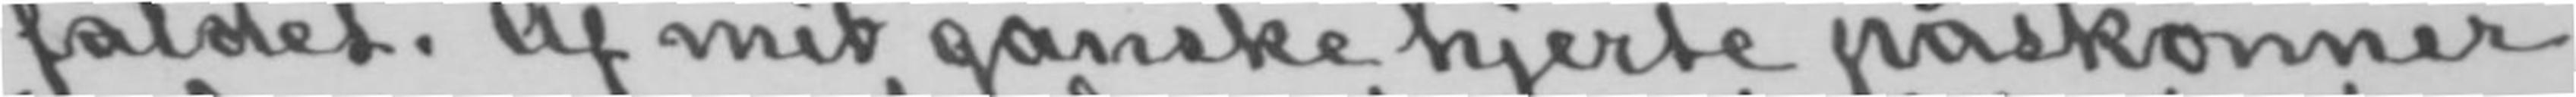faldet. Af mit ganske hjerte påskønner
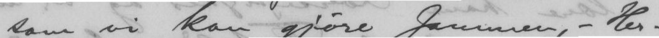som vi kan gjøre sammen, - Her-
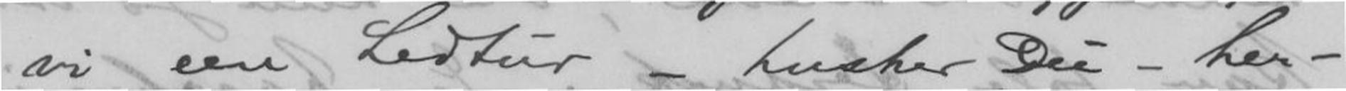vi een Ledtur - husker Du - her-
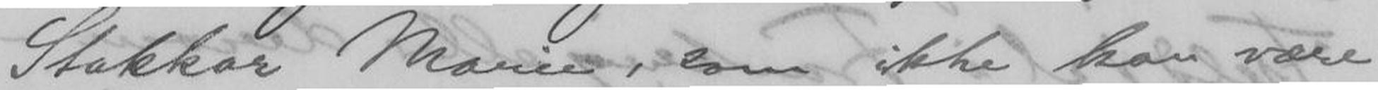Stakkar Marie, som ikke kan være
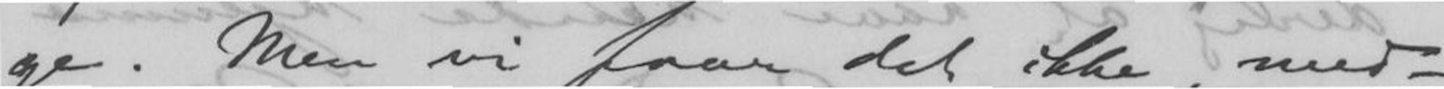ge. Men vi faar det ikke, med-
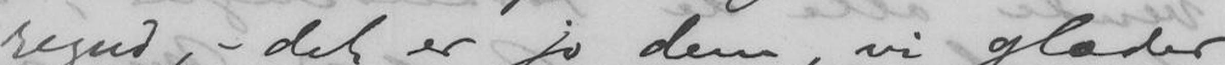regud, - det er jo dem, vi glæder
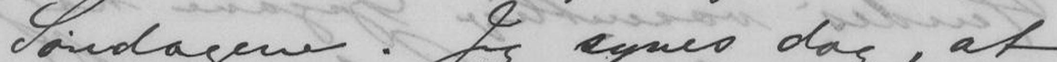Søndagene. Jeg synes dog, at
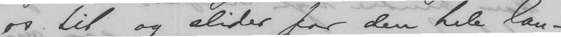os til og slider for den hele lan-
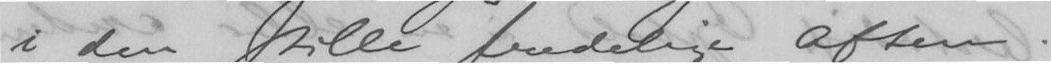i den stille fredelige Aften.
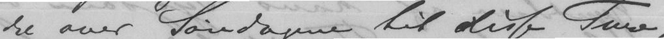re over Søndagene til disse Ture,
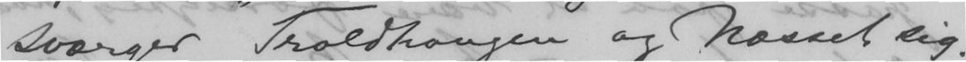sværger Troldhaugen og Næsset sig.
